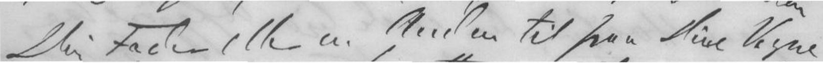Din Fader eller en Anden til paa Dine Vegne
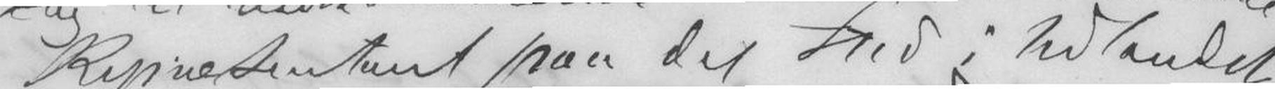Representant paa det Sted i Udlandet
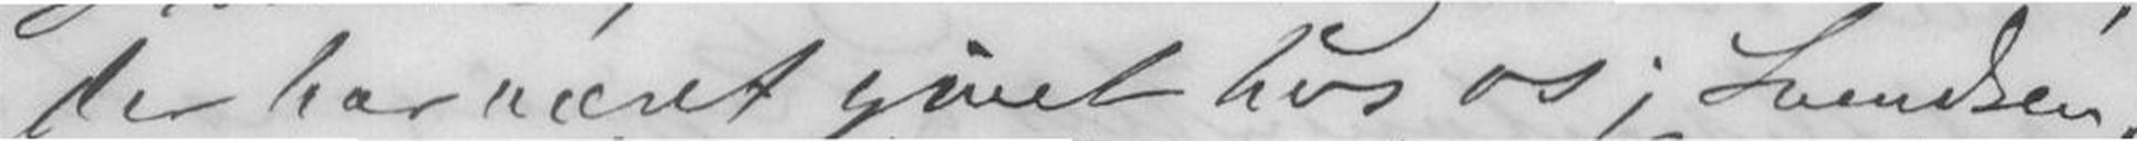der har været givet hos os, Svendsen,
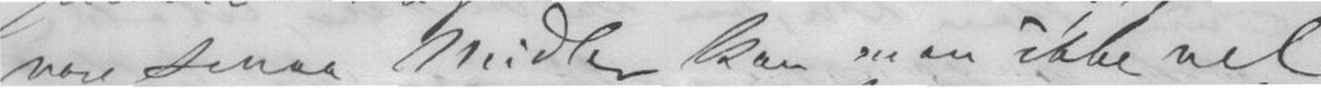vore smaa Midler kan man ikke vel
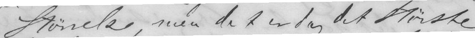Størrelse, men det er dog det Største,
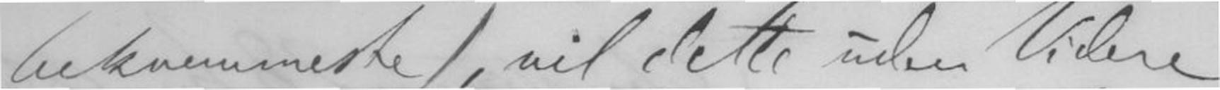bekvemmeste) vil dette uden Videre
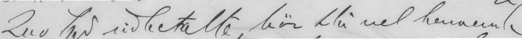200 Spd udbetalte, bør Du vel henvende
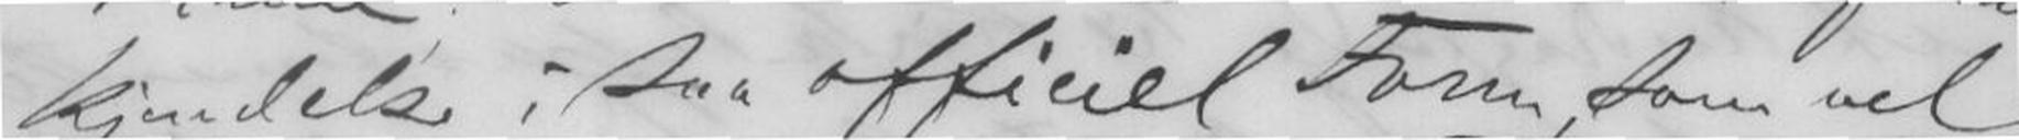kjendelse i saa officiel Form, som vel
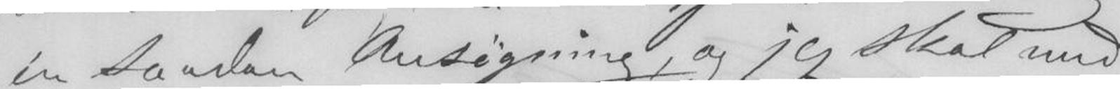én saadan Ansøgning, og jeg skal med
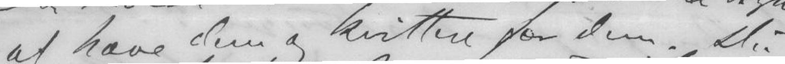at hæve dem og kvittere for Dem. Du
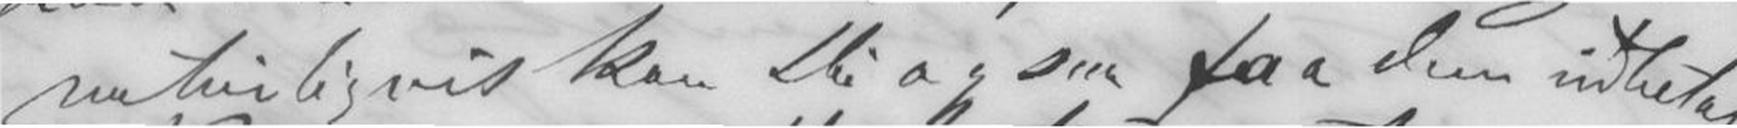naturligvis kan Du ogsaa faa dem udbetalte
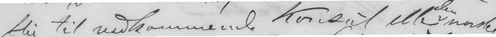Dig til vedkommende Konsul eller norske
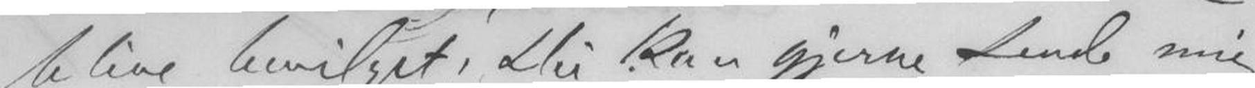blive bevilget, Du kan gjerne sende mig
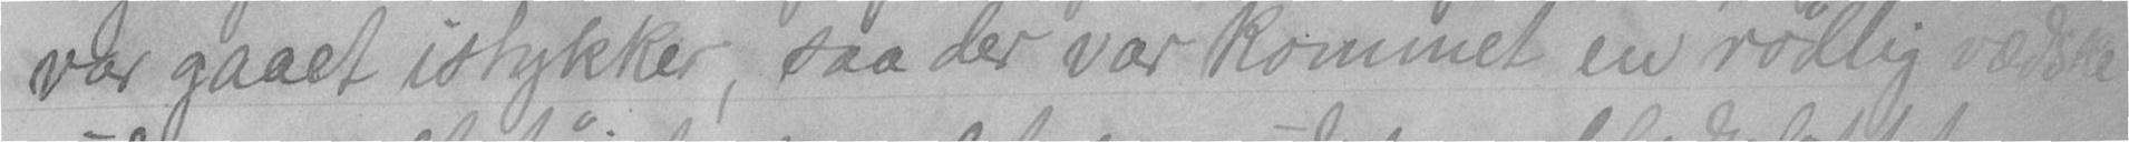var gaaet istykker, saa der var kommet en rødlig vædske
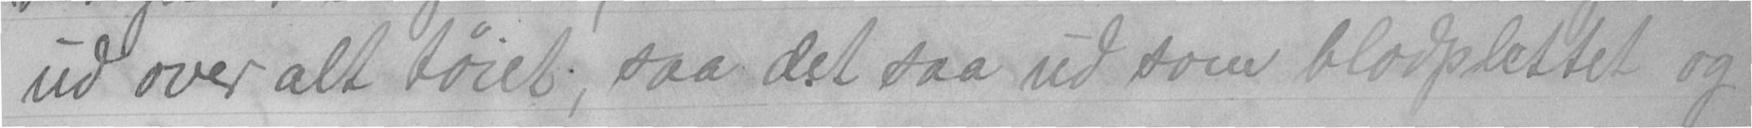ud over alt tøiet; saa det saa ud som blodplettet og
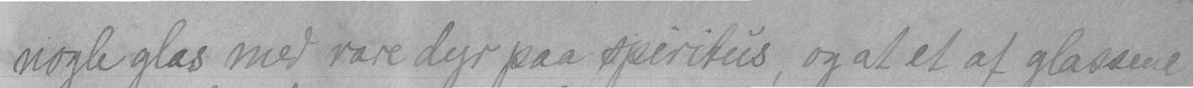nogle glas med rare dyr paa spiritus, og at et af glassene
